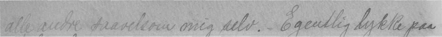alle andre saavelsom mig selv. - Egentlig lykke paa
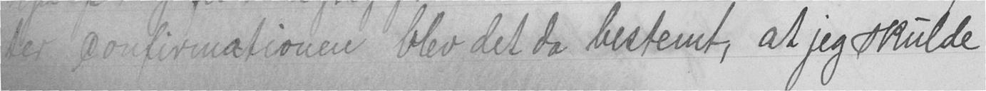ter confirmationen blev det da bestemt, at jeg skulde
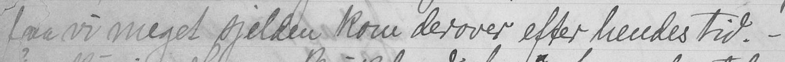saa vi meget sjelden kom derover efter hendes tid. -
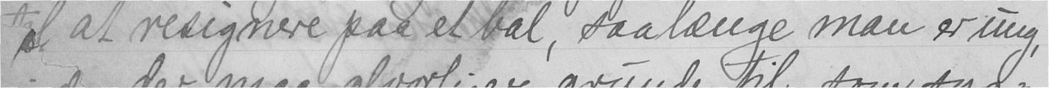til at resignere paa et bal, saa længe man er ung,
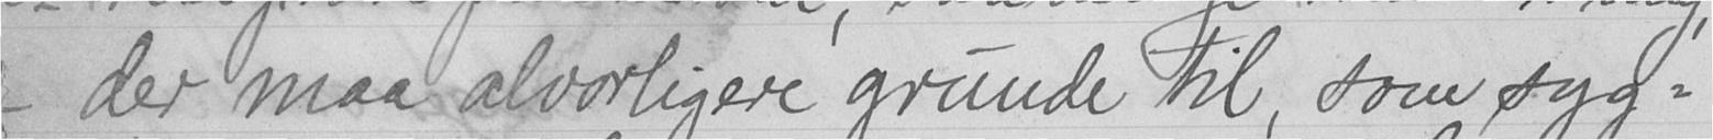der maa alvorligere grunde til, som syg-
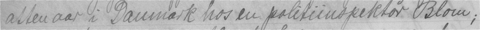atten aar i Danmark hos en politiinspektør Blom;
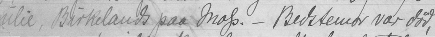milie, Birkelands paa Mafs. - Bedstemor var død,
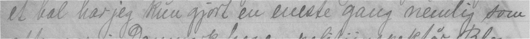et bal har jeg kun gjort en eneste gang nemlig som
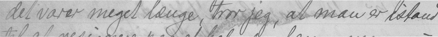det varer meget længe tror jeg, at man er istand
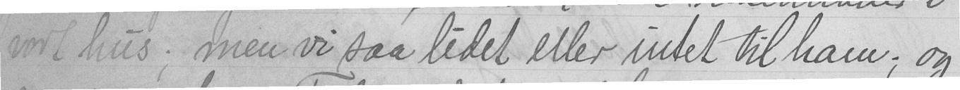vort hus; men vi saa lidet eller intet til ham; og
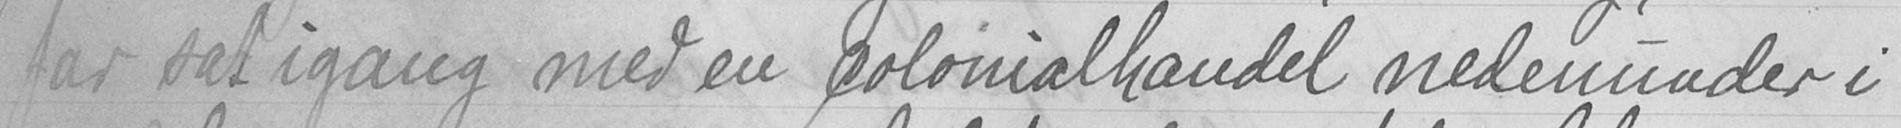far sat igang med en colonialhandel nedenunder i
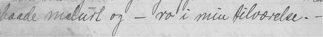baade malurt og - ro i min tilværelse. -
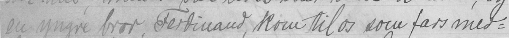en yngre bror, Ferdinand, kom til os som fars med-
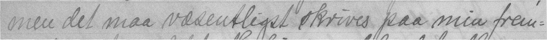men det maa væsentligst skrives paa min frem-
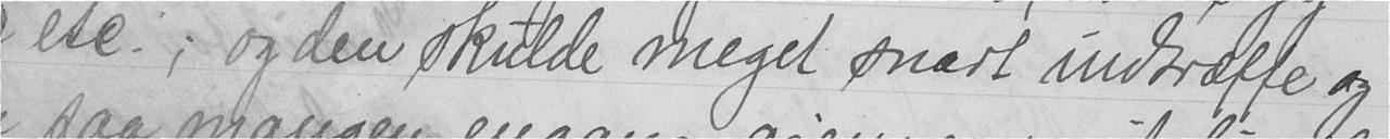dom etc.; og den skulde meget snart indtræffe og
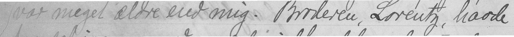var meget ældre end mig. Broderen, Lorentz, havde
